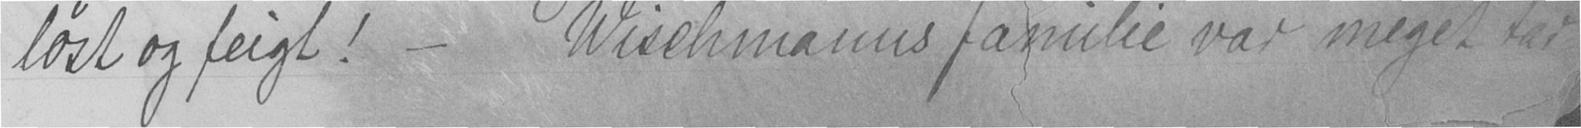løst og feigt! - Wischmanns familie var meget tar-
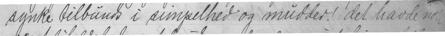synke tilbunds i simpelhed og mudder! det havde nok
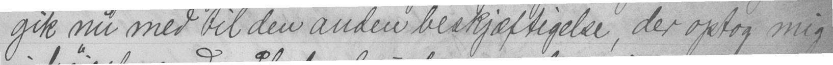gik nu med til den anden beskjæftigelse, der optog mig
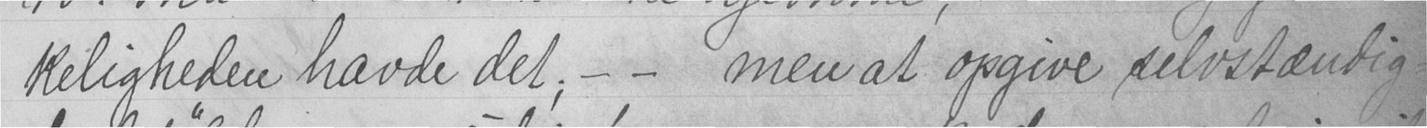keligheden havde det; - - men at opgive selvstændig-
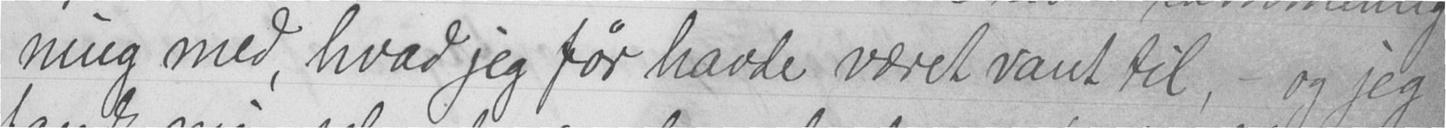ning med, hvad jeg før havde været vant til, - og jeg
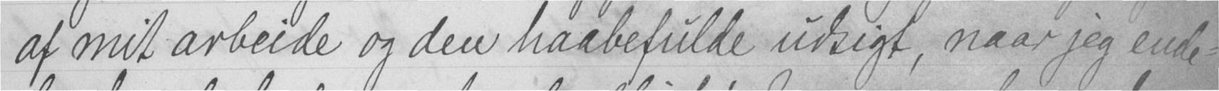af mit arbeide og den haabefulde udsigt, naar jeg ende-
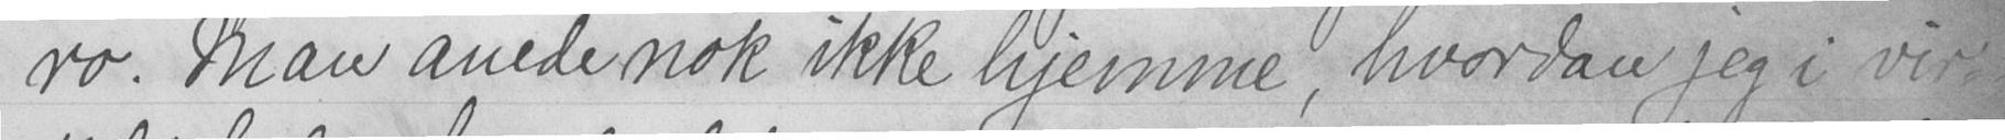ro. Man anede nok ikke hjemme, hvordan jeg i vir-
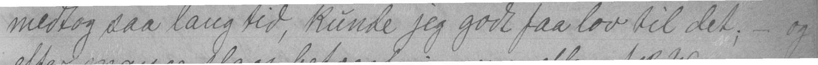medtog saa lang tid, kunde jeg godt faa lov til det; - og
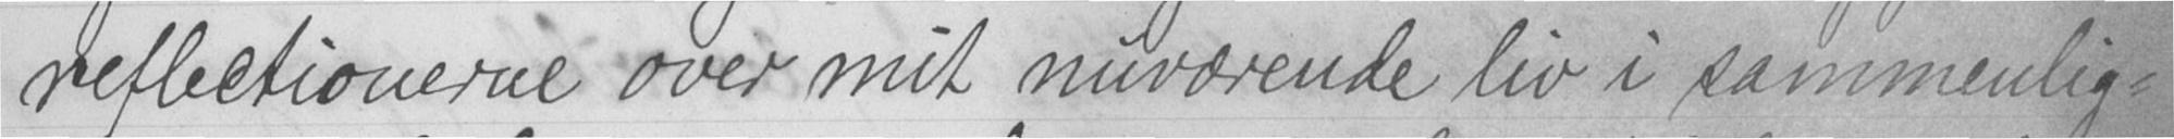reflectionerne over mit nuværende liv i sammenlig-
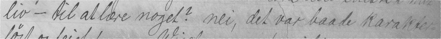liv - til at lære noget? nei, det var baade karakter-
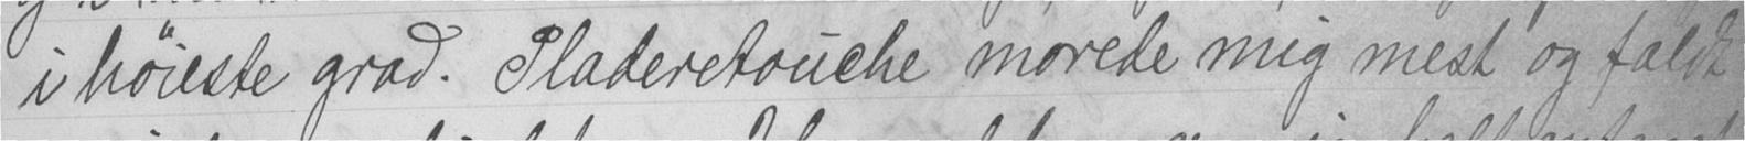i høieste grad. Pladeretouche morede mig mest og faldt
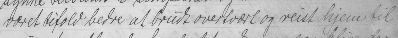været tifold bedre at brudt overtvært og reist hjem til
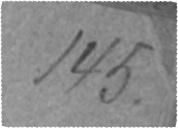145.
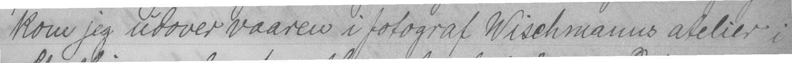kom jeg udover vaaren i fotograf Wischmanns atelier i
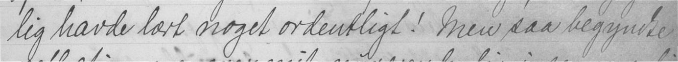lig havde lært noget ordentligt! Men saa begyndte
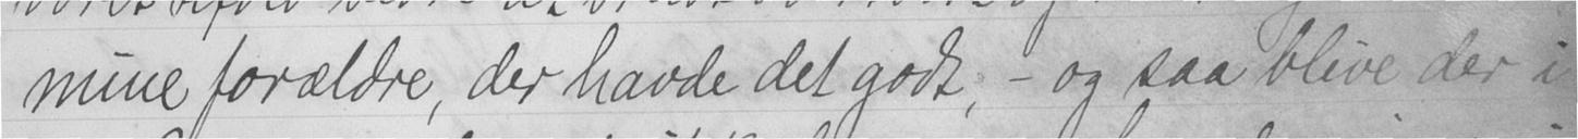mine forældre, der havde det godt, - og saa blive der i
